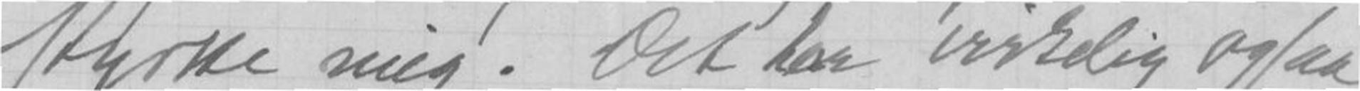styrke mig. Det har virkelig ogsaa
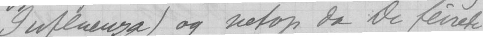Influenza) og netop da De feiret
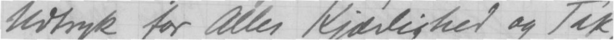Udtryk for Alles Kjærlighed og Tak-
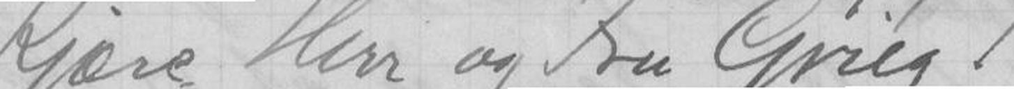Kjære Herr og Fru Grieg!
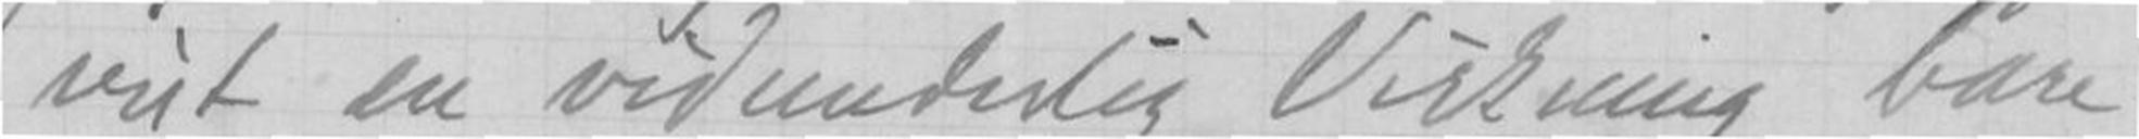vist en vidunderlig Virkning bare
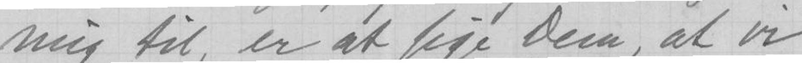mig til, er at sige dem at vi
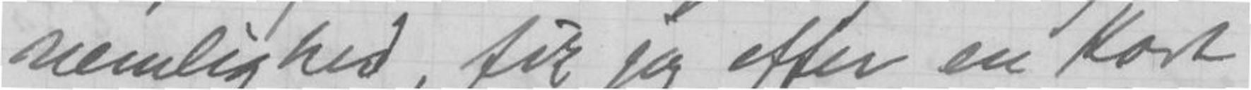nemlighed, fik jeg efter en Kort
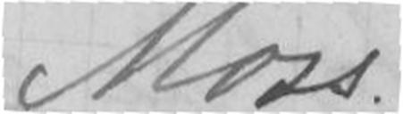Moss
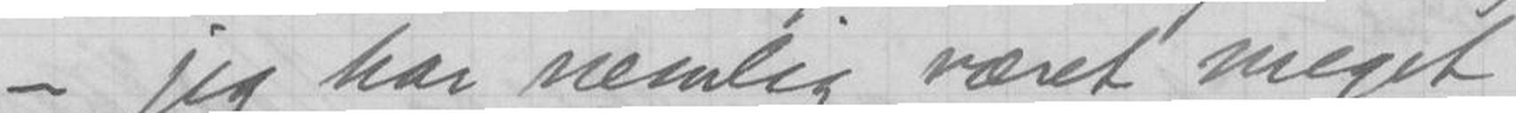- jeg har nemlig været meget
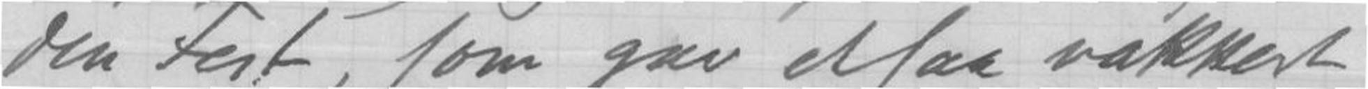Den Fest, som gav et saa vakkert
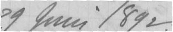29 Juni 1892.
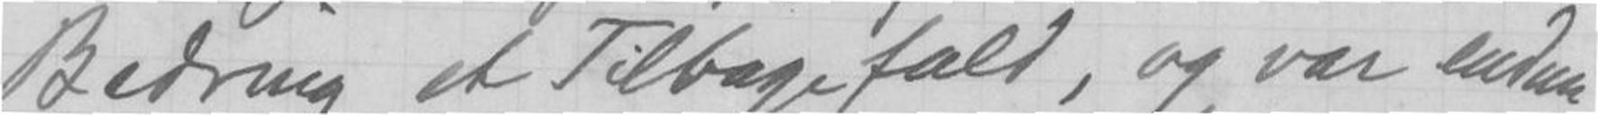Bedring et Tilbagefald, og var endnu
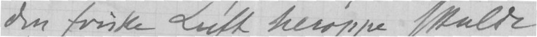den friske Luft heroppe skulde
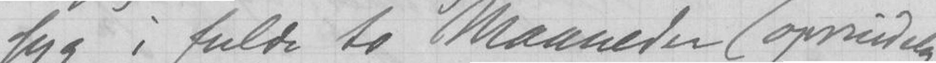Syg i fulde to maaneder (oprindelig
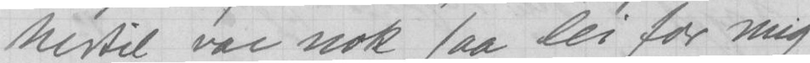hertil var nok saa lei for mig
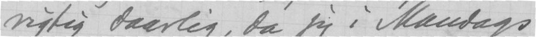rigtig daarlig, da jeg i Mandags
